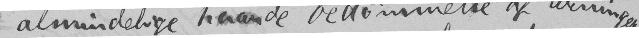almindelige haarde bedømmelse af urninger-
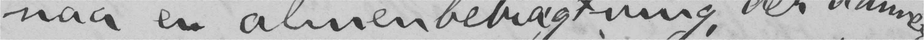naa en almenbetragtning, der danner
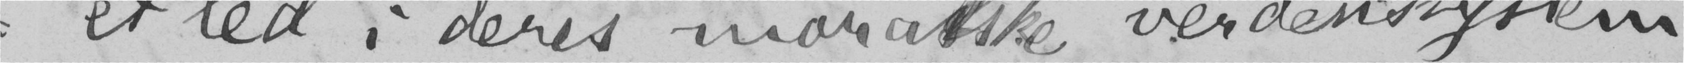et led i deres moralske verdenssystem.
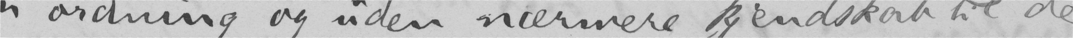ordning og uden nærmere kjendskab til de
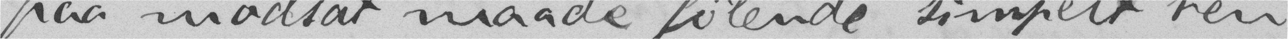paa modsat maade følende, simpelt hen
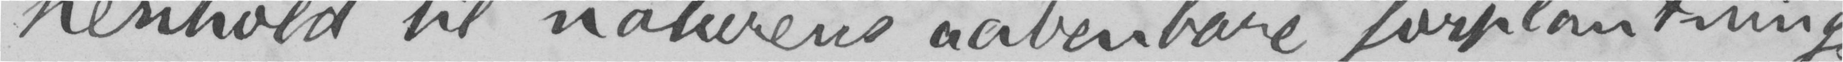henhold til naturens aabenbare forplantnings-
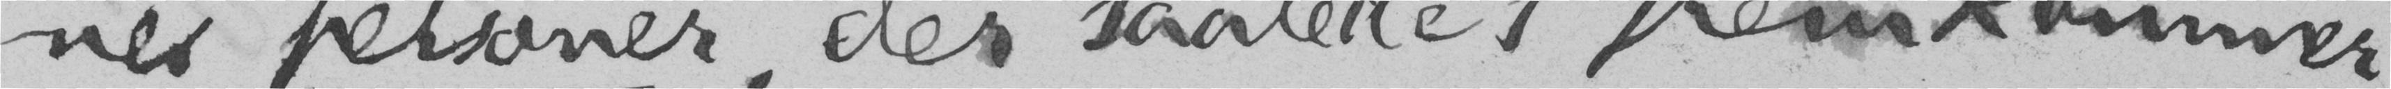nes personer, der saaledes fremkommer,
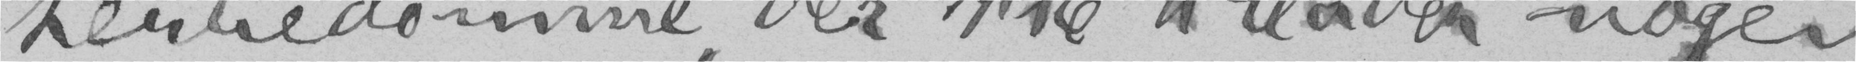herredømme, der ikke tillader nogen
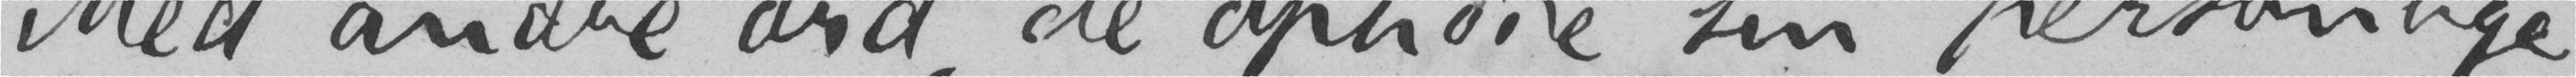Med andre ord, de ophøie sin personlige
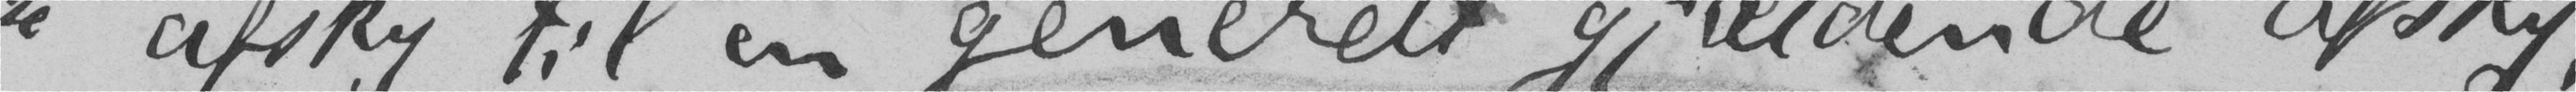afsky til en generelt gjældende afsky,
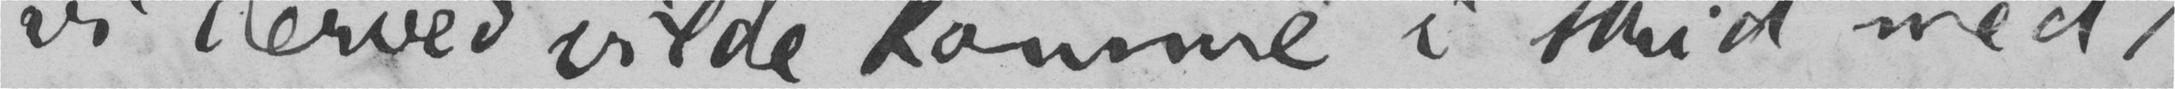vi derved vilde komme i strid med
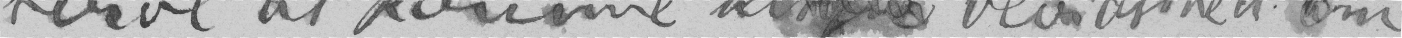tvivl at komme til <….??> bevidsthet om,
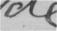de
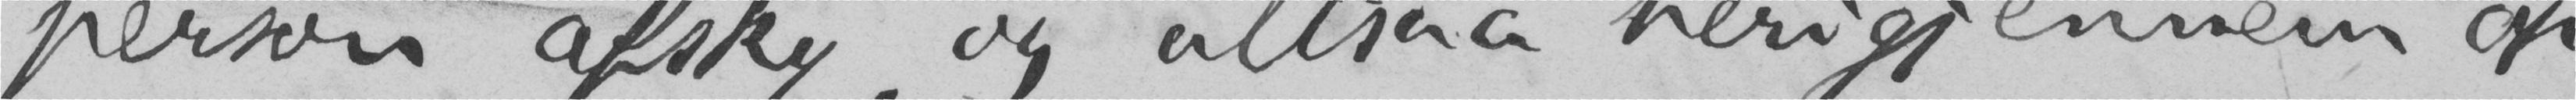person afsky, og altsaa herigjennem op-
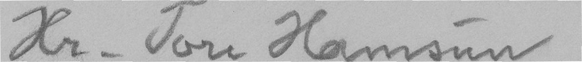Hr. Tore Hamsun
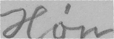Høn
